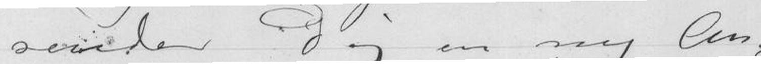og sender Dig en ny An-
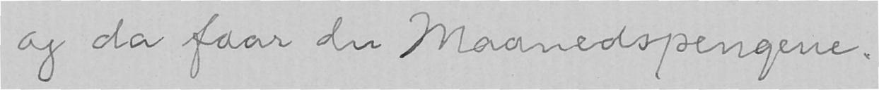og da faar du Maanedspengene.
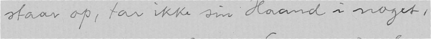staar op, tar ikke sin Haand i noget,
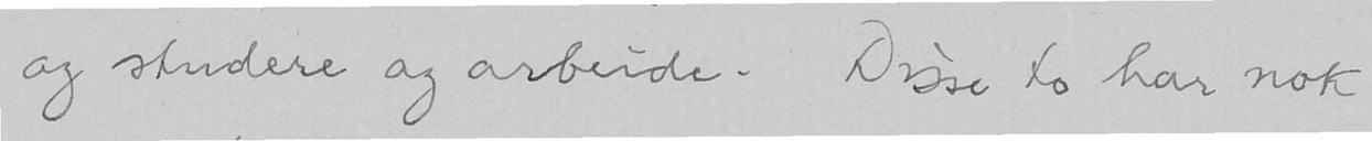og studere og arbeide. Disse to har nok
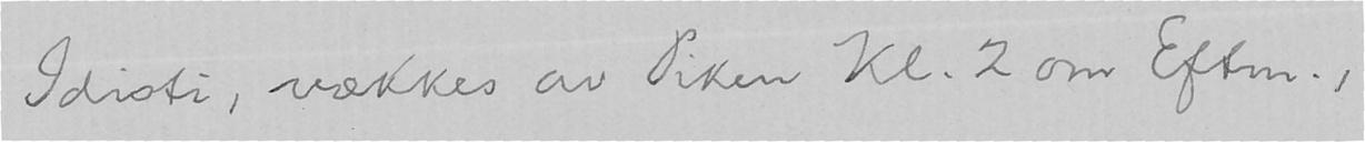Idioti, vækkes av Piken Kl. 2 om Eftm.,
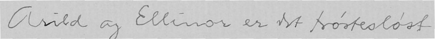Arild og Ellinor er det trøstesløst
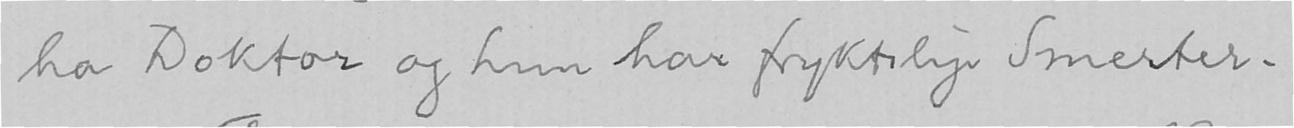ha Doktor og hun har fryktelige Smerter.
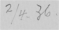2/4. 36.
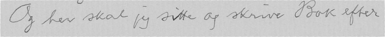Og her skal jeg sitte og skrive Bok efter
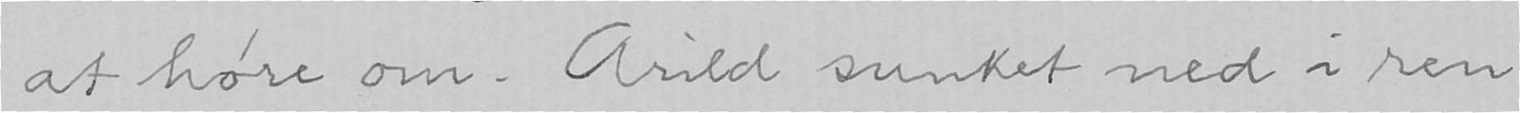at høre om. Arild sunket ned i ren
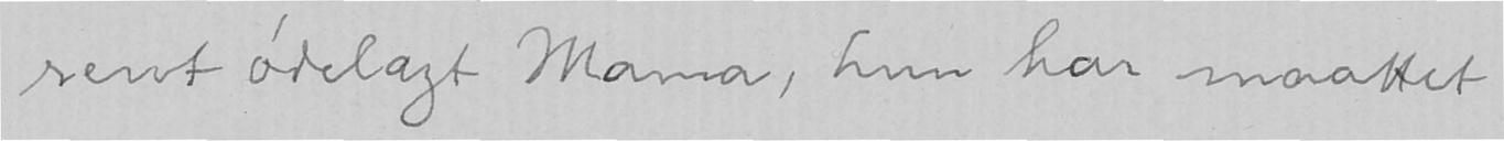rent ødelagt Mama, hun har maattet
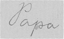Papa
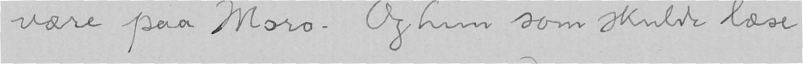være paa Moro. Og hun som skulde læse
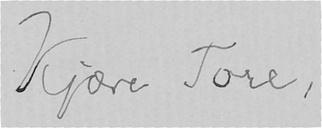Kjære Tore,
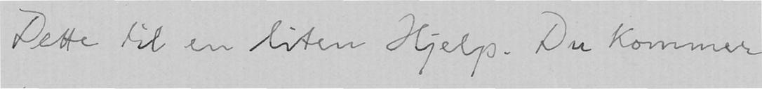Dette til en liten Hjelp. Du kommer
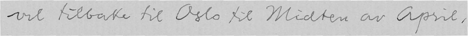vel tilbake til Oslo til Midten av April,
